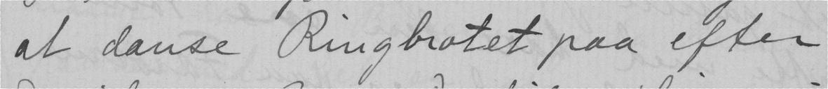at danse Ringbrotet paa efter
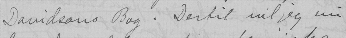Davidsons Bog. Dertil vil jeg nu
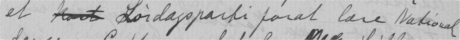et kort Lørdagsparti forat lære National-
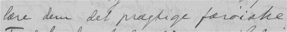lære dem det prægtige færøiske
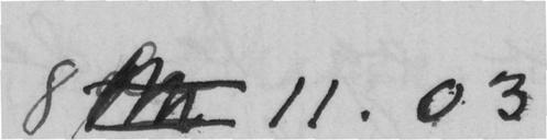8  11.03
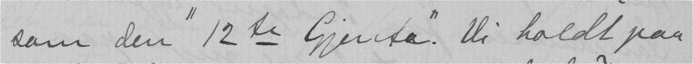som den "12te Gjenta". Vi holdt paa
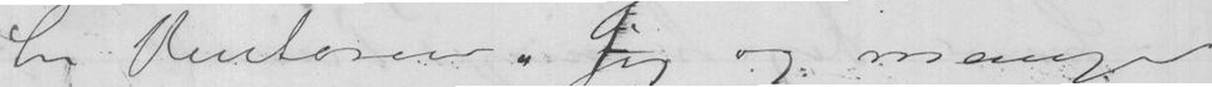til Vinteren. Jeg og mange
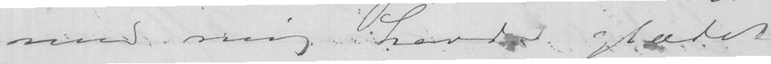med mig havde glædet
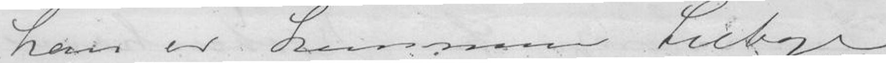han er kommen tilbage
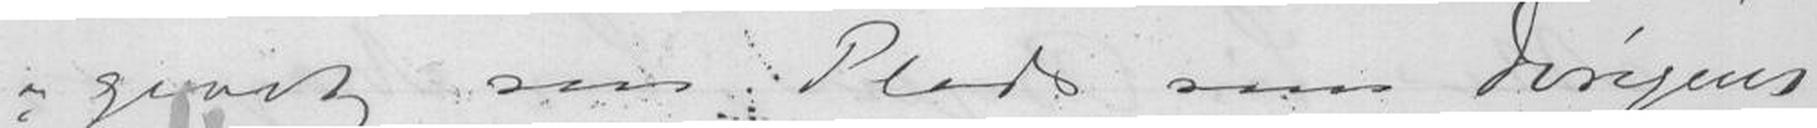givet sin Plads som Dirigent
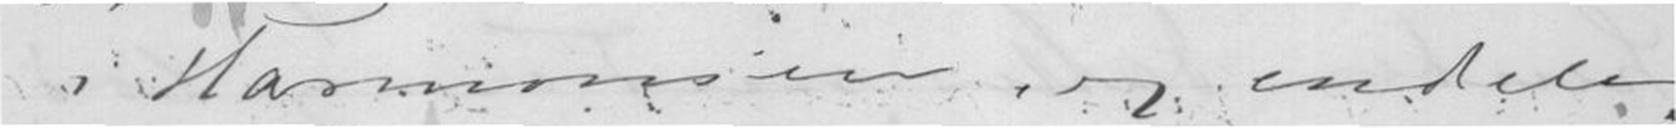i Harmonien og endelig
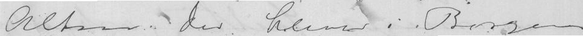Altsaa Du bliver i Bergen
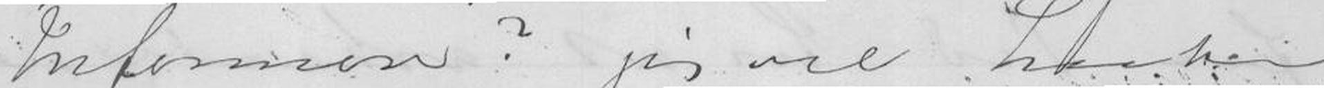Informere? Jeg vil haabe
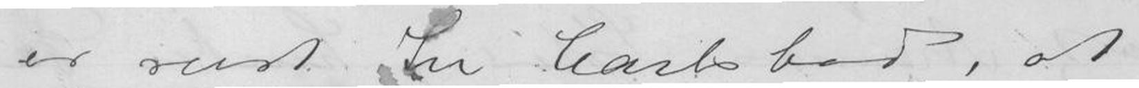er reist til Carlsbad, at
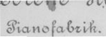Pianofabrik.
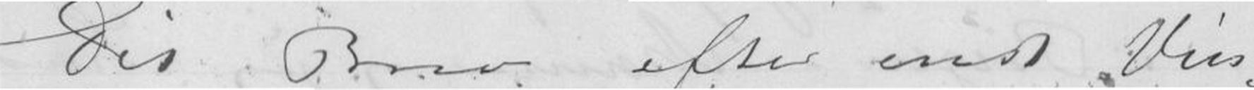Dit Brev efter endt Vin
